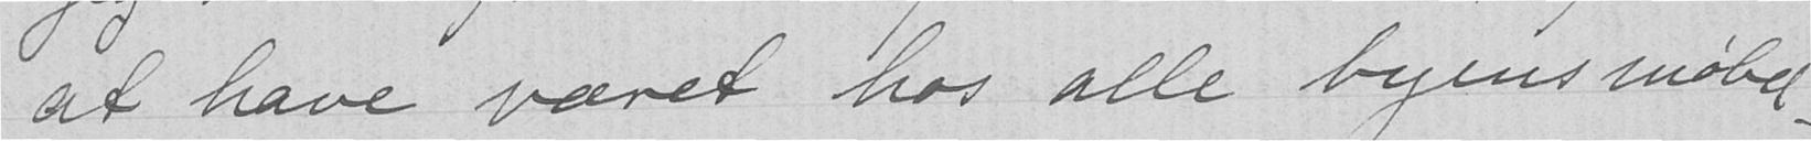at have været hos alle byens møbel-
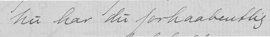Nu har du forhaabentlig
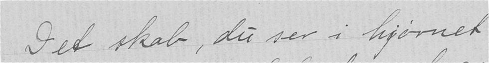- Det skab, du ser i hjørnet
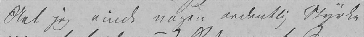Skal jeg vinde nogen ordentlig Styrke
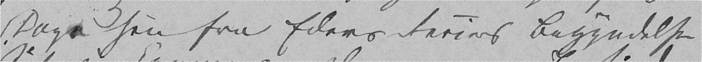Dage hen fra Eders Feriers Begyndelse
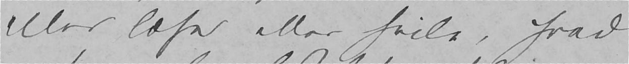eller læse eller hvile, hvad
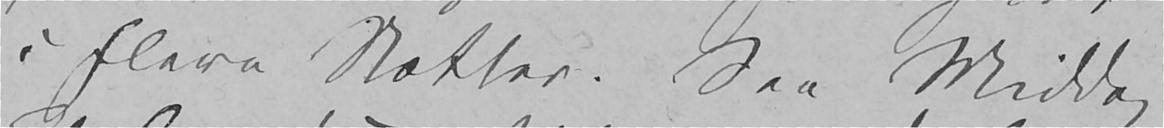i flere Nætter. Saa Middag
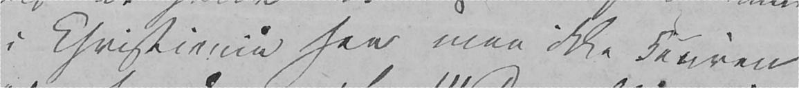i Christiania saa maa ikke Couren
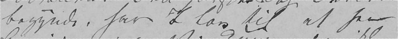begynde, have I Lov til at see
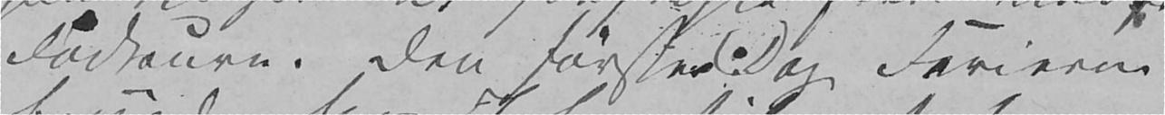Fodtoure. Den første Dag Ferierne
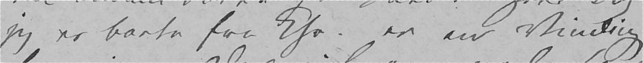jeg er borte fra Chr. er en Vinding
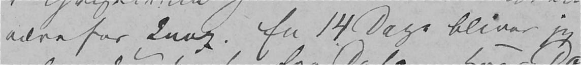være for knap. En 14 Dage bliver jeg
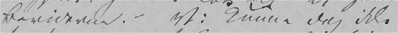Beriderne. - Vi kunne dog ikke
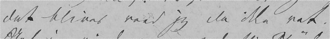det bliver veed jeg da ikke ret.
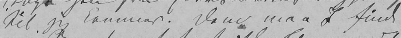til jeg kommer. Dem maa I finde
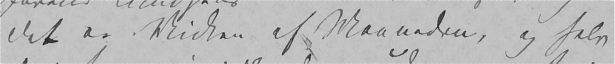det er Midten af Maaneden, og selv
